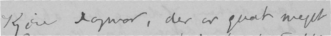Kjære Dagmar, der er gaat svært meget
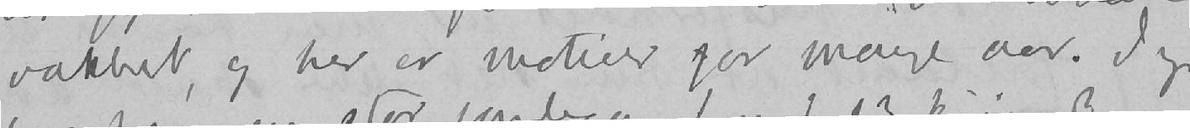vakkert, og her er motiv for mange aar. Jeg
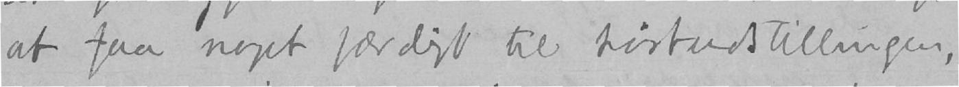at faa noget færdigt til høstudstillingen,
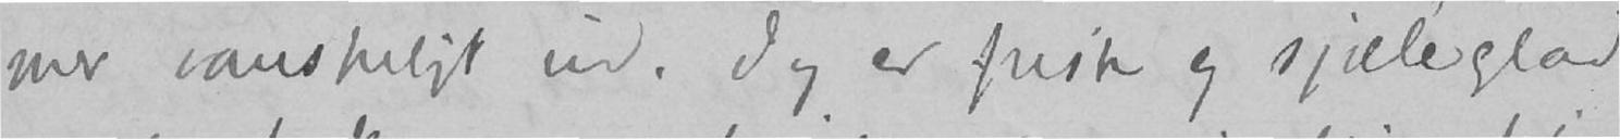mer vanskeligt ind. Jeg er frisk og sjæleglad
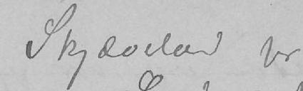Skjæveland pr
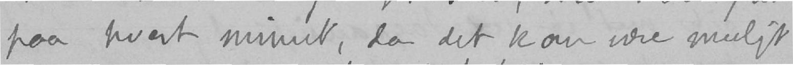paa hvert minut, da det kan være muligt
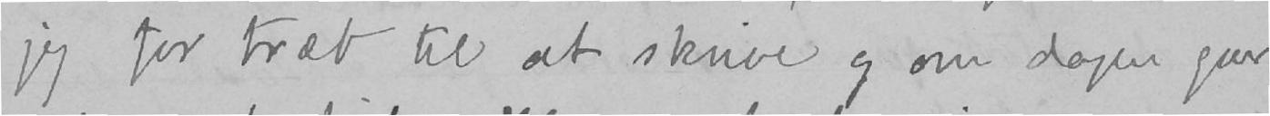jeg for træt til at skrive og om dagen gaar
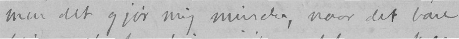men det gjør mig mindre, naar det bare
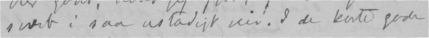svært i saa ustadigt veir. I de korte gode
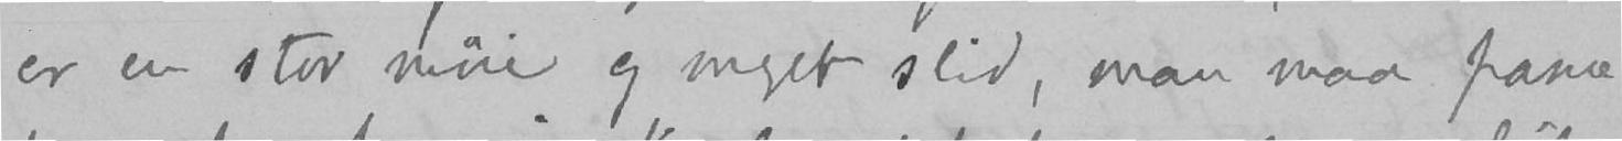er en stor møie og meget slid, man maa passe
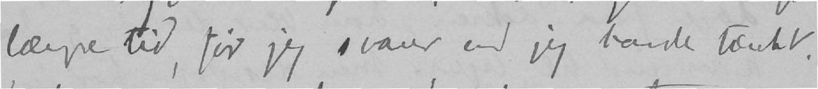længre tid, før jeg svarer end jeg havde tænkt.
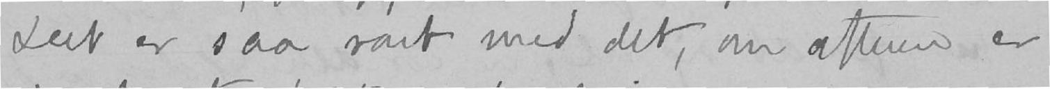Det er saa rart med det, om aftenen er
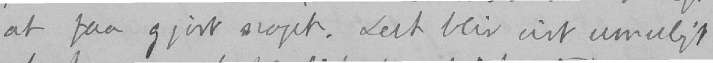at faa gjort noget. Det blir vist umuligt
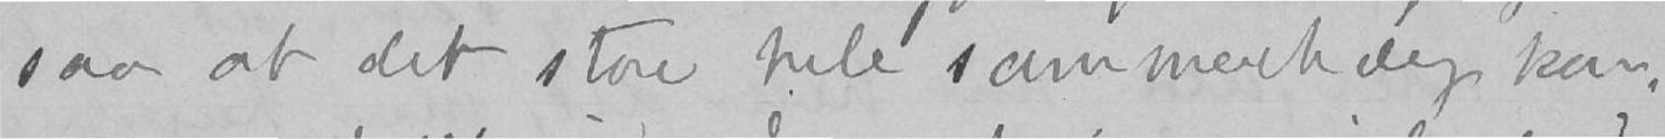saa at det store hele sammenhæng kom-
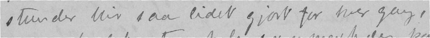stunder blir saa lidet gjort for hver gang,
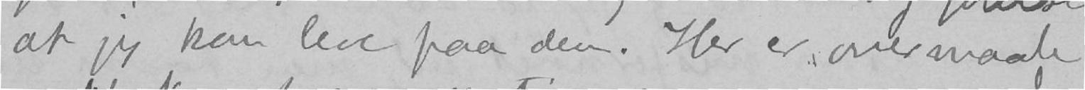at jeg kan leve paa den. Her er overmaade
